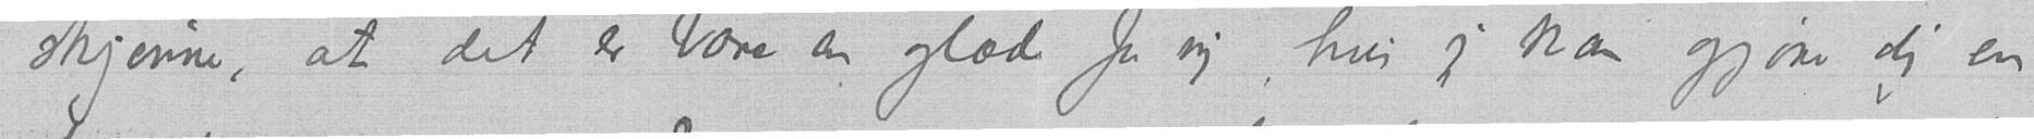skjønne, at det er bare en glæde for mig, hvis jeg kan gjøre dig en
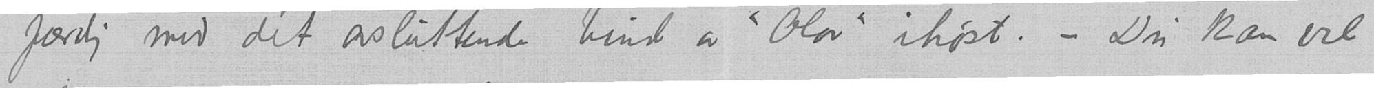færdig med det avsluttende bind av «Olav» ihøst. – Du kan vel
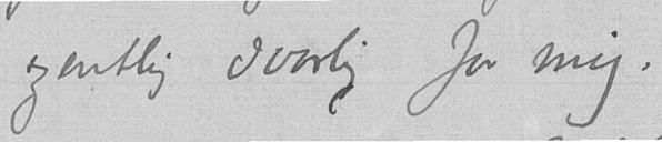egentlig daarlig for mig.
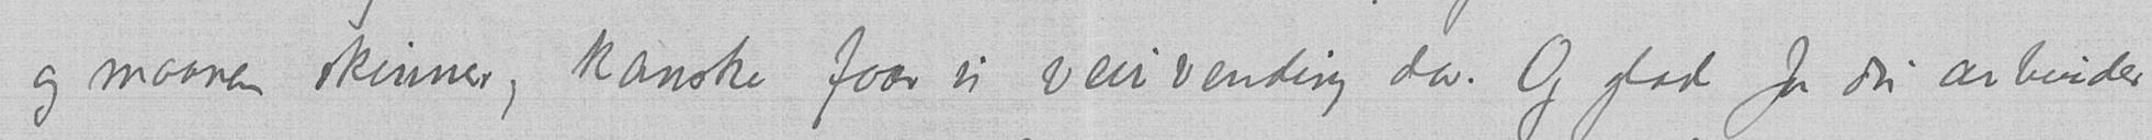og maanen skinner, kanske faar vi veirvending da. Og glad for du arbeider
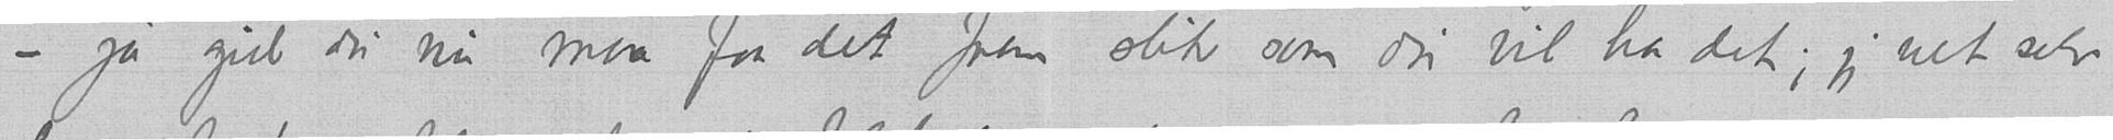– ja gid du nu maa faa det frem slik som du vil ha det; jeg vet selv
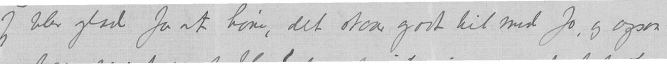Jeg blev glad for at høre, det staar godt til med Jo, og ogsaa
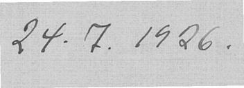24.7.1926.
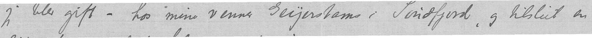jeg blev gift – hos mine venner Geijerstams i Sundfjord, og tilslut en
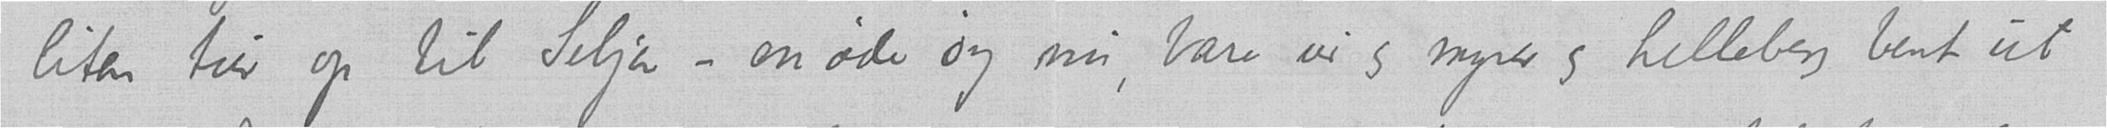liten tur op til Selje – en øde øy nu, bare ur og myrer og helleberg bent ut
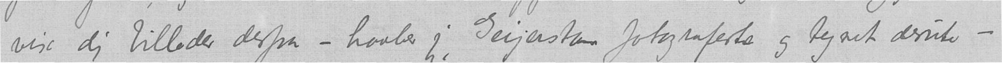vise dig billeder derfra – haaber jeg, Geijerstams fotograferte og tegnet derute –
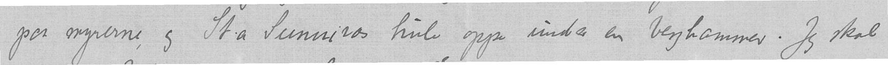paa myrerne, og St.a Sunnivas hule oppe under en berghammer. Jeg skal
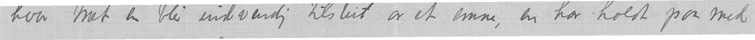hvor træt en blir indvendig tilslut av et emne, en har holdt paa med
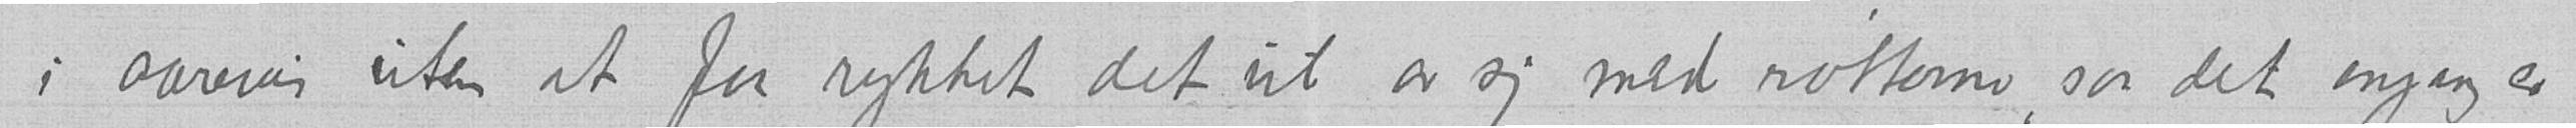i aarevis uten at faa rykket det ut av sig med røtterne, saa det engang er
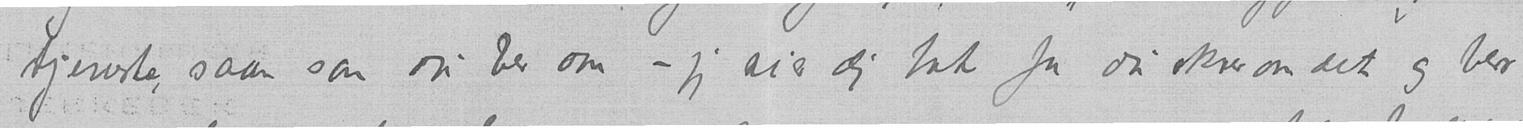tjeneste, saan som du ber om – jeg sier dig tak for du skrev om det og ber
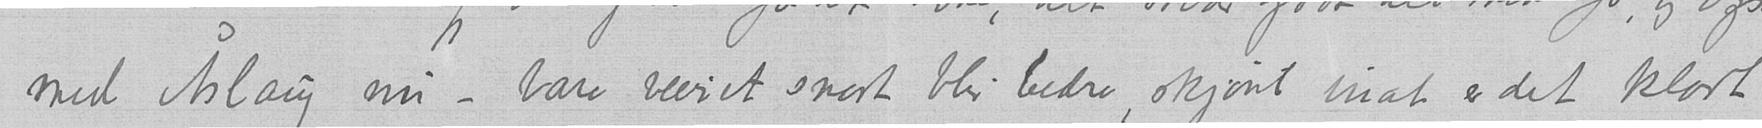med Åslaug nu – bare veiret snart blir bedre, skjønt inat er det klart
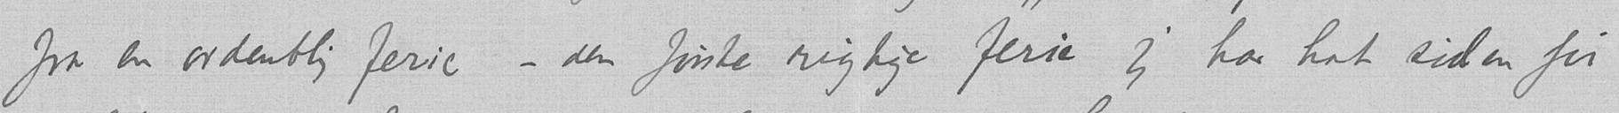fra en ordentlig ferie – den første rigtige ferie jeg har hat siden før
